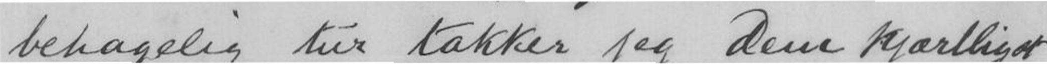behagelig tur takker jeg Dem kjærligst
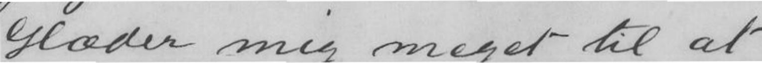Glæder mig meget til at
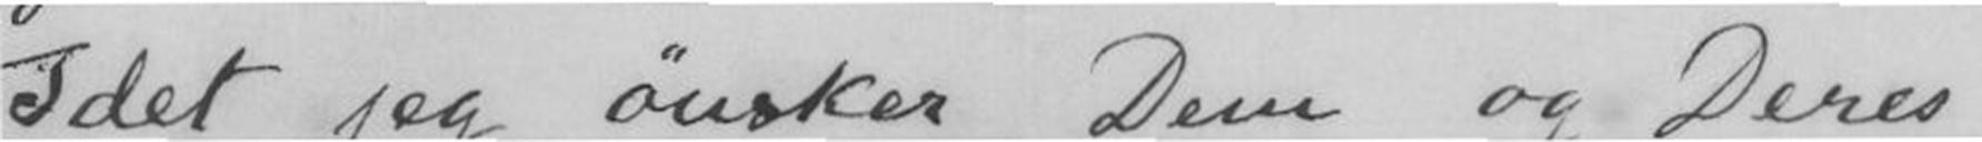Idet jeg ønsker Dem og Deres
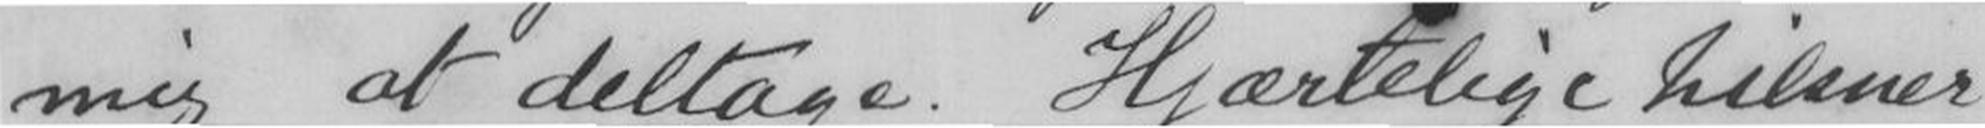mig at deltage. Hjærtelige hilsner
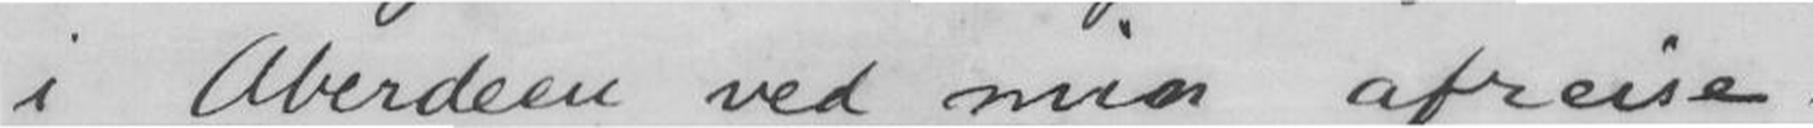i Aberdeen ved min afreise.
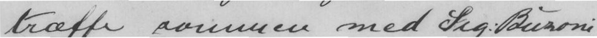træffe sammen med Sig.Buzoni
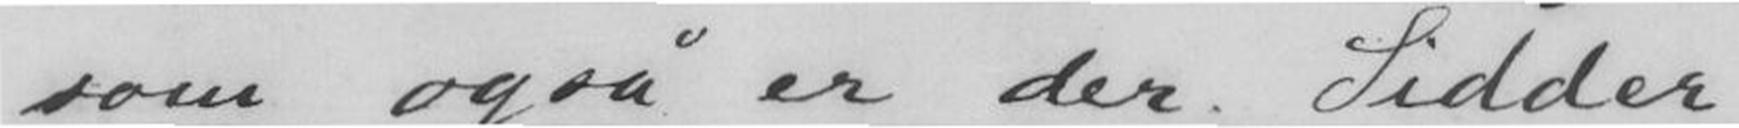som også er der. Sidder
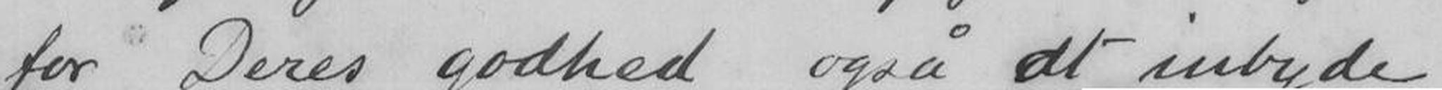for Deres godhed også at inbyde
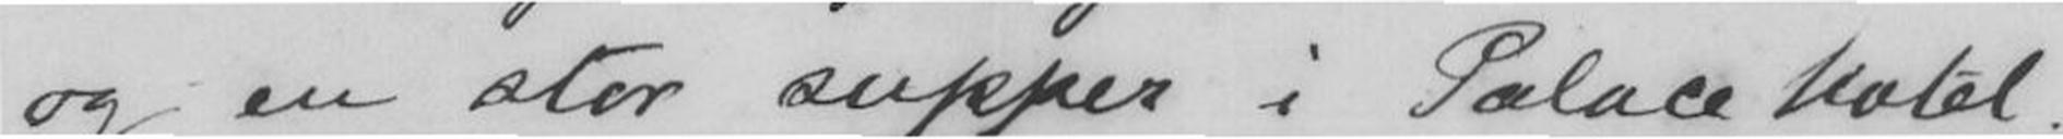og en store supper i Palace hotel.
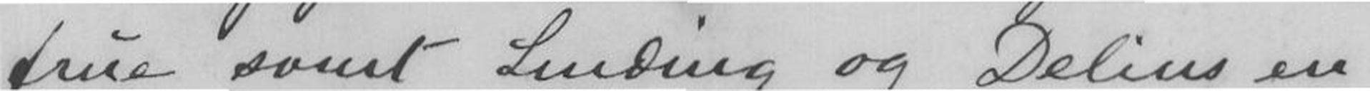frue samt Sinding og Delius en
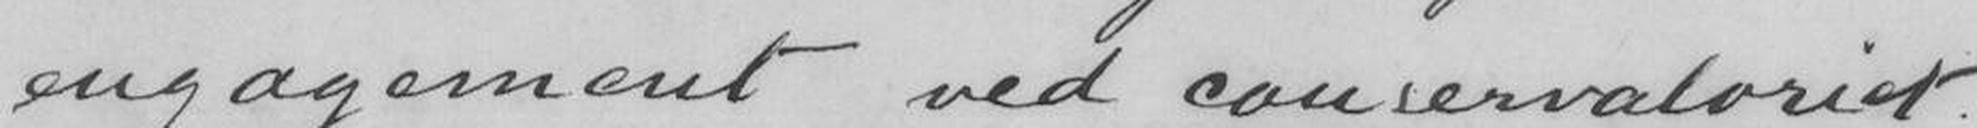engagement ved conservatoriet.
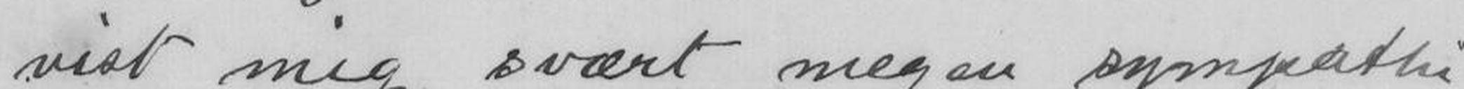vist mig svært megen sympathi
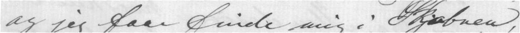og jeg faae finde mig i Skjæbnen,
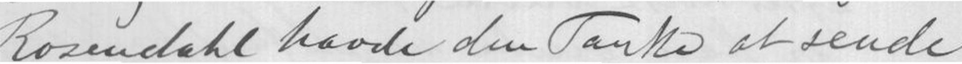Rosendahl havde den Tanke at sende
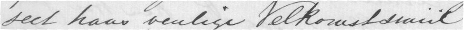seet hans venlige Velkomstsmil
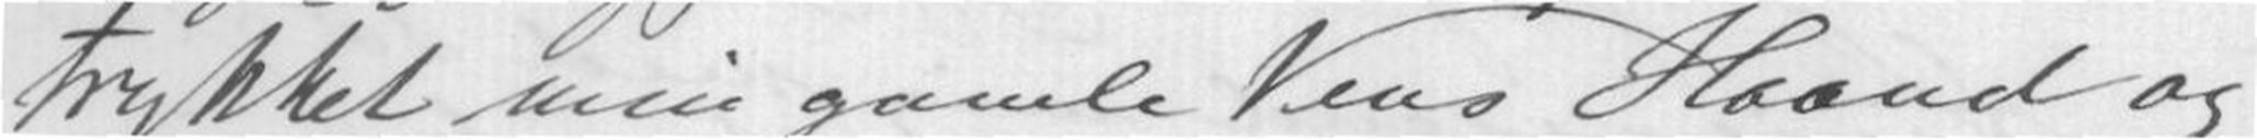trykket min Vens haand og
